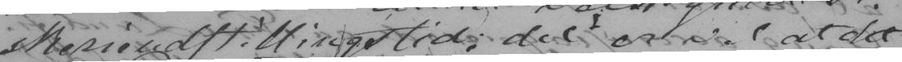kerieudstillingstid, det er vel at det
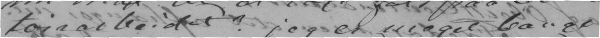toirarbeidet? jeg er meget bange
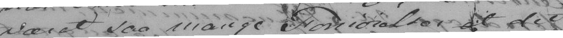været saa mange Fornøielser at det
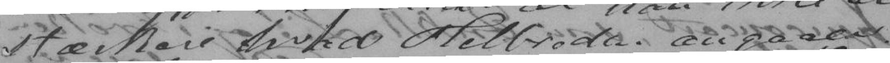stærkere hvad Helbreden angaaer.
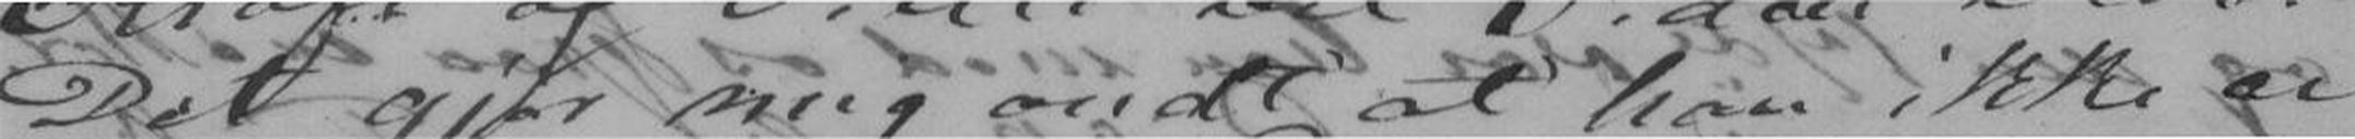Det gjør mig ondt at han ikke er
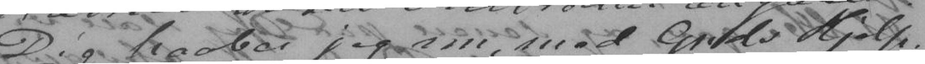Dig haaber jeg nu, med Guds Hjelp,
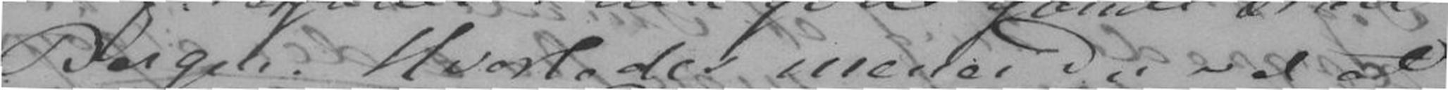Bergen. Hvorledes mener Du vel at
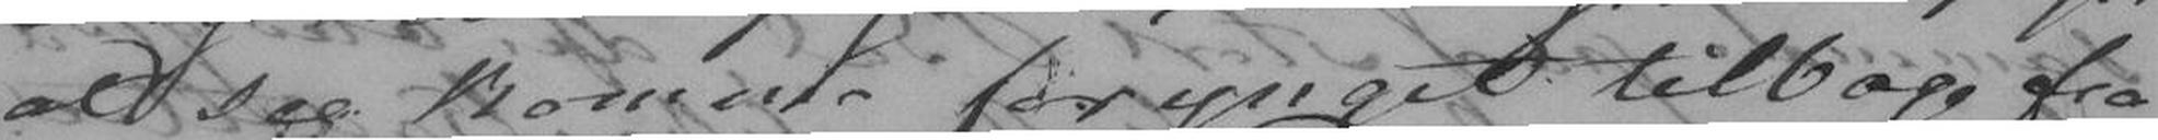at see komme forynget tilbage fra
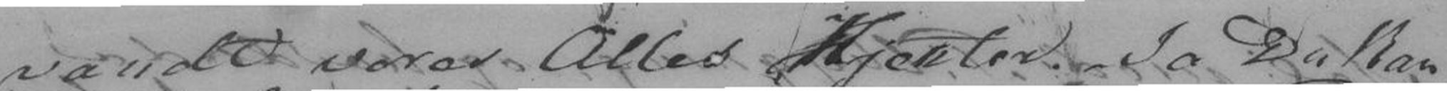ndt vores Alles Hjerter, Ja Du kan
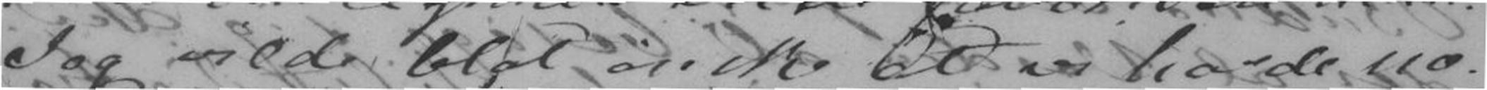Jeg vilde blot ønske at vi havde no-
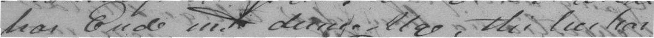har Ende med denne Uge, thi her har
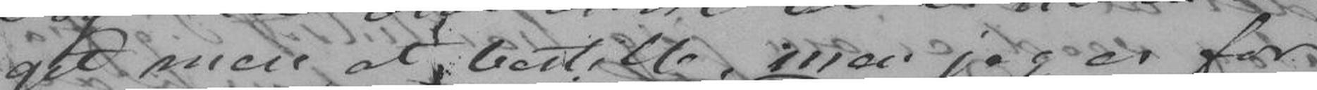get mere at bestille, men jeg er for
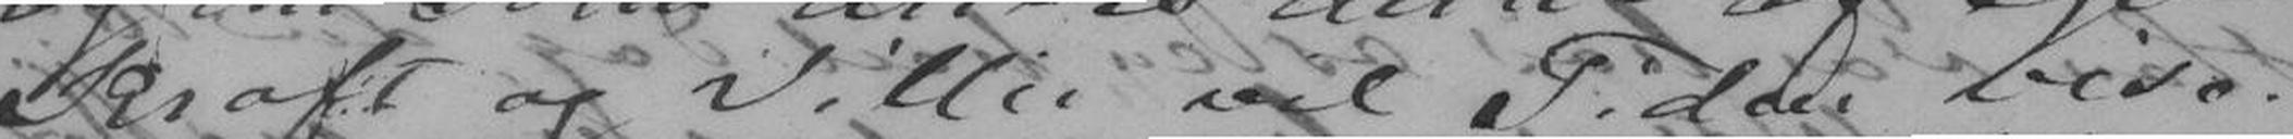Kraft og Villie vil Tiden vise.
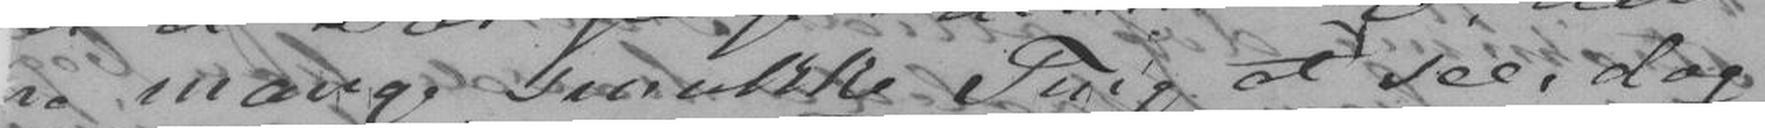re mange Smukke Ting at see, dog
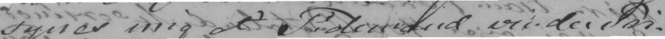synes mig at Tidemand vinder Pri-
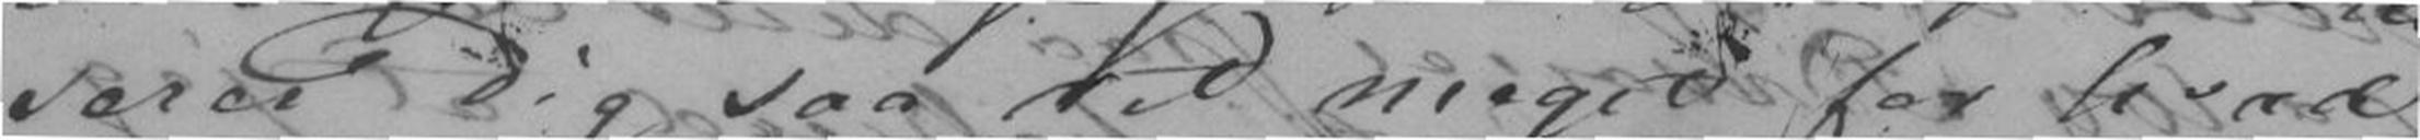serer Dig saa ret meget for hvad
